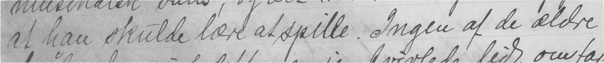at han skulde lære at spille. Ingen af de ældre
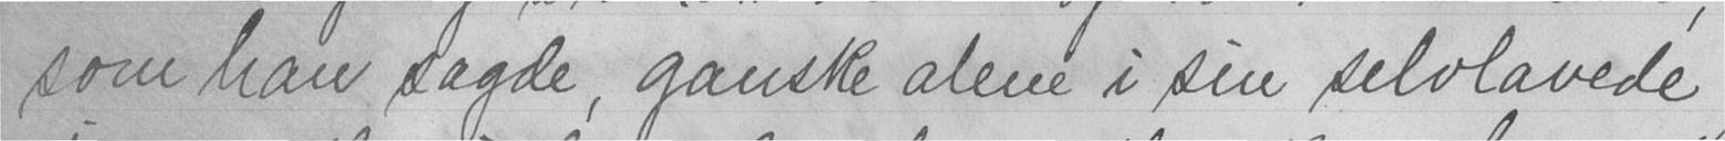som han sagde, ganske alene i sin selvlavede
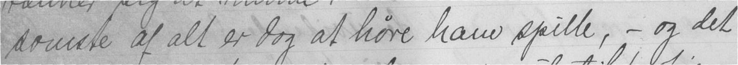somste af alt er dog at høre ham spille, - og det
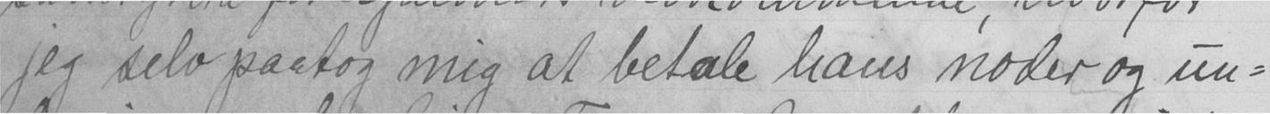jeg selv paatog mig at betale hans noder og un-
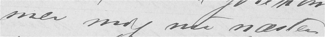mer mig nu næsten
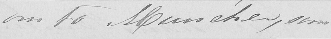om to Muncher, som
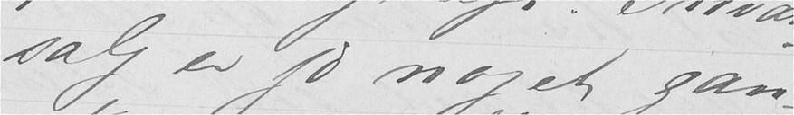salg er jo noget gan-
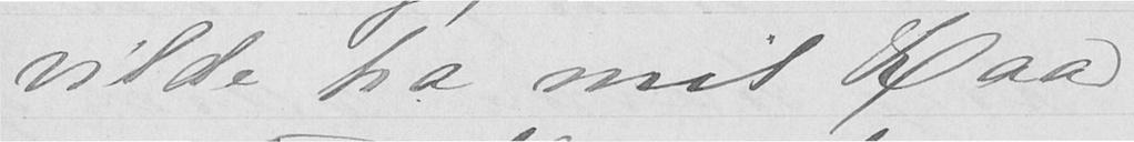vilde ha mit Raad
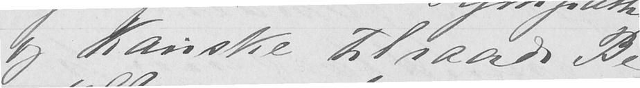og kanske tilraade Be-
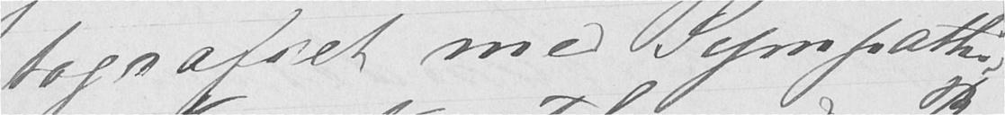tografiet med Sympathi
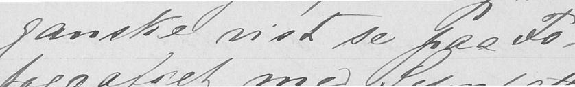ganske vist se paa Fo-
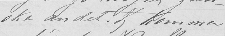ske andet. Jeg kommer
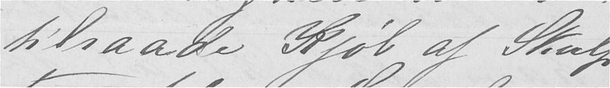tilraade kjøb af Skulp-
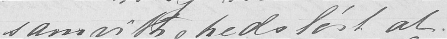samvittighedsløst at
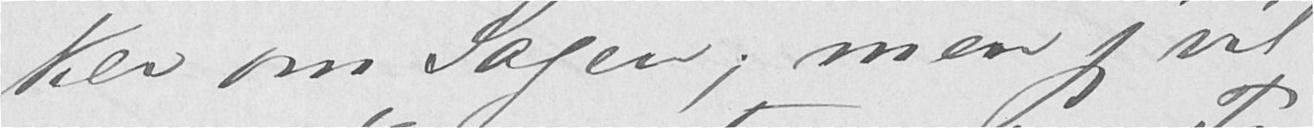ker om Sagen; men jeg vil
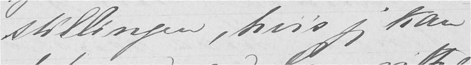stillingen, hvis jeg kan
